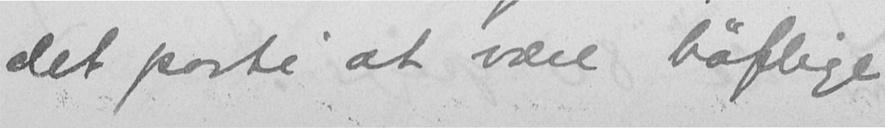det parti at være høflige
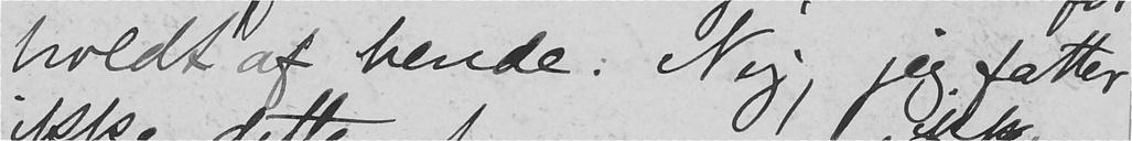holdt af hende. Nej, jeg fatter
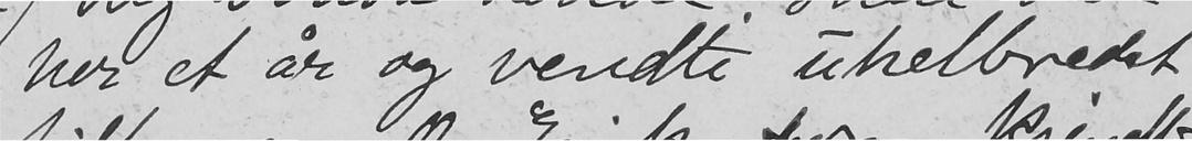her et år og vendte uhelbredet
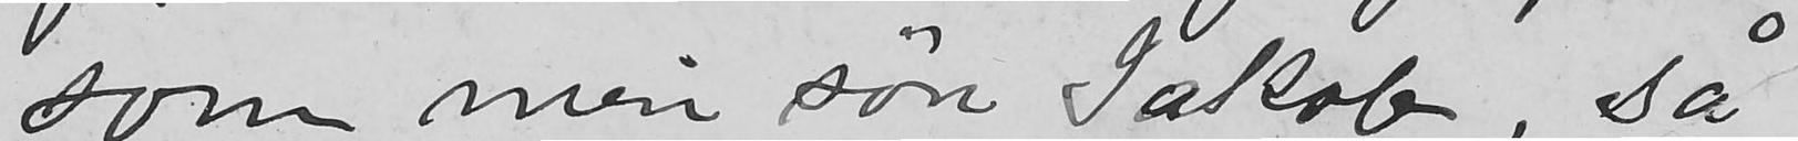som min søn Jakob, så
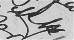ikke
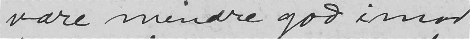være mendre god imod
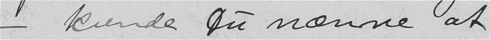- kunde Du nænne at
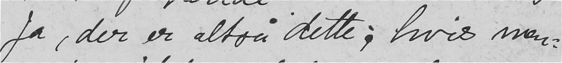Ja, der er altså dette; hvad men-
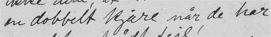en dobbelt kjære når de har
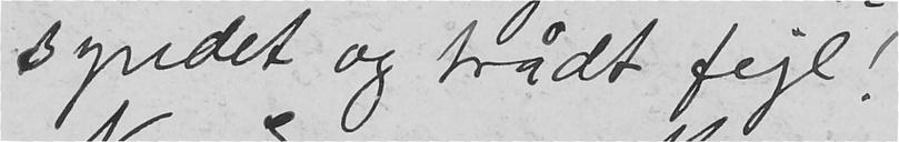syndet og trådt fejl!
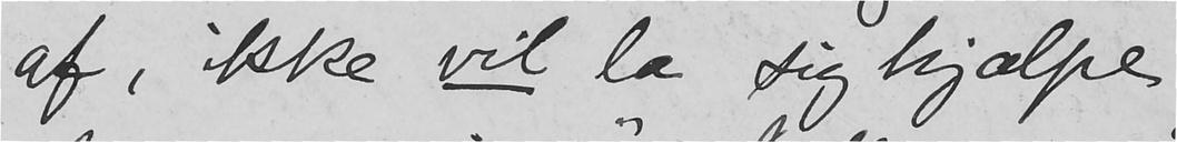af, ikke vil la si hjælpe
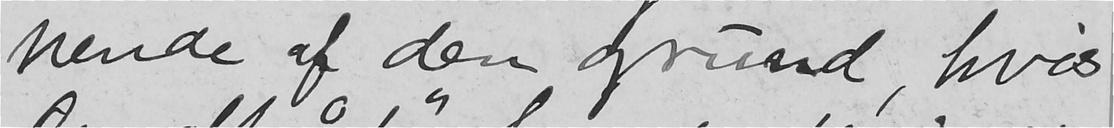hende af den grund, hvis
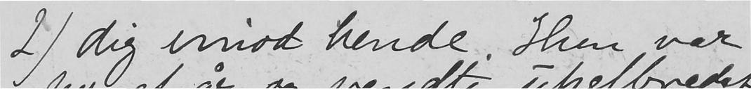2) dig imod hende. Hun var
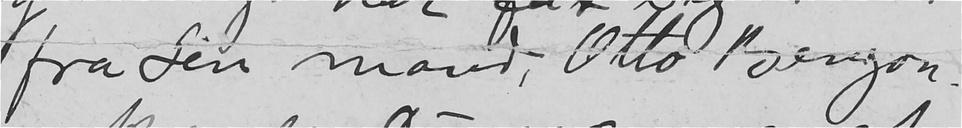fra sin mand, Otto Benzon.
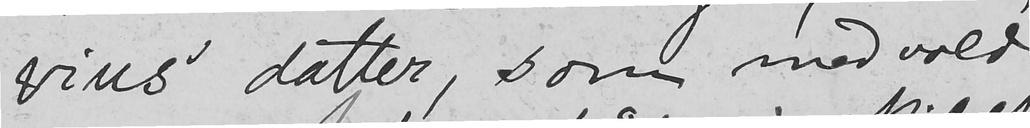vius' datter, som med vold
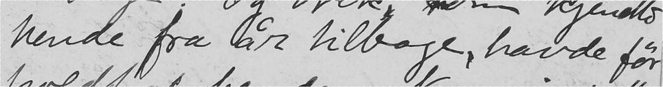hende fra år tilbage, havde før
